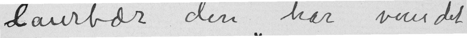laurbær den har vundet
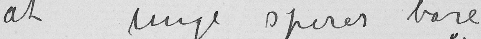at unge spirer bare
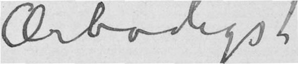Ærbødigst
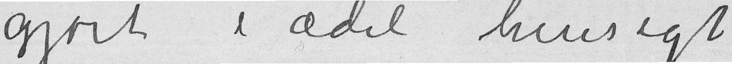gjort i ædel hensigt
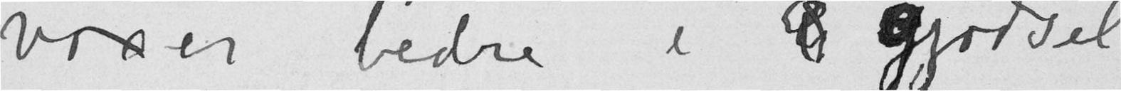voxer bedre i gjødsel
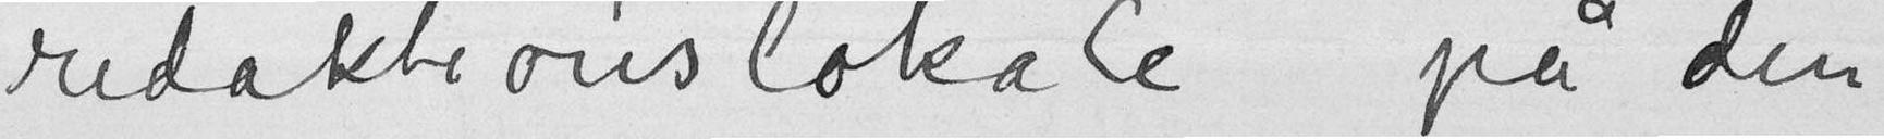redaktionslokale på den
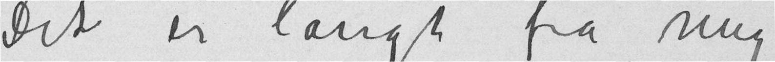Det er langt fra mig
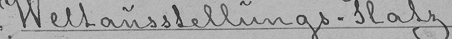Weltausstellungs-Platz.
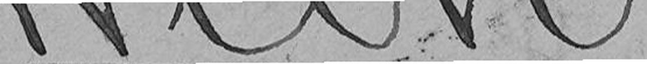Wien.
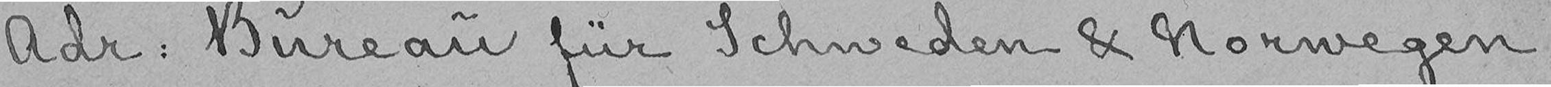Adr: Bureau für Schweden & Norwegen.
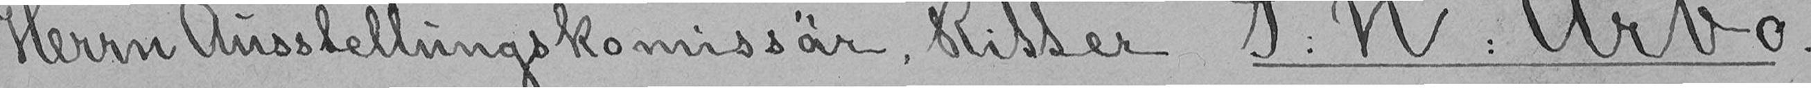Herrn Ausstellungskomissär, Ritter P: N: Arbo.
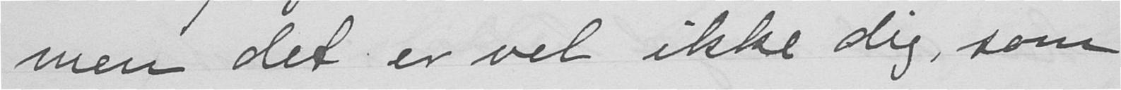men det er vel ikke dig, som
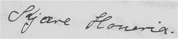Kjære Honoria.
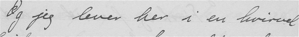Og jeg lever her i en hvirvel
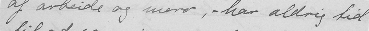af arbeide og moro ,- har aldrig tid
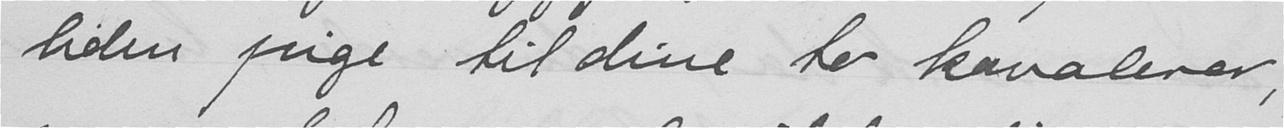liden pige til dine to kavalerer.
